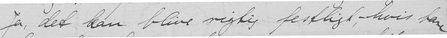Ja, det kan blive rigtig festligt, - hvis bare
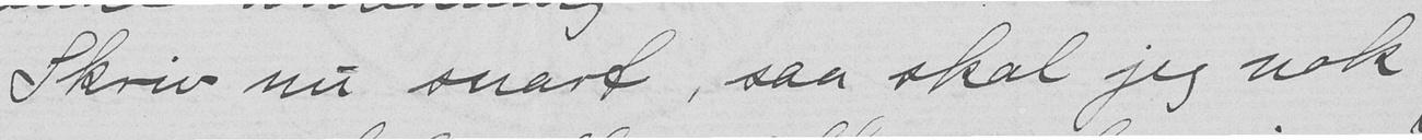Skriv nu snart, saa skal jeg nok
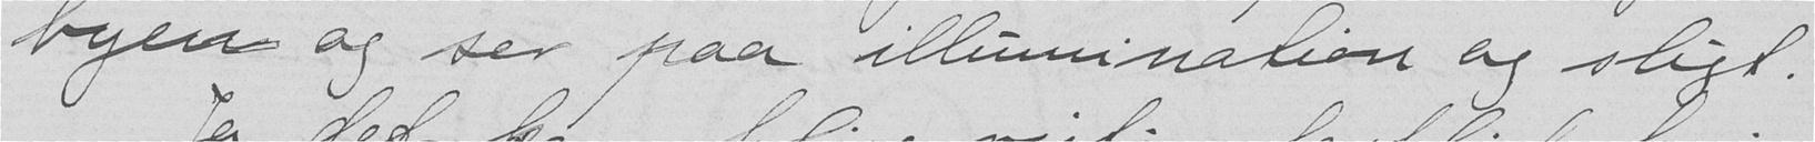byen og ser paa illumination og sligt.
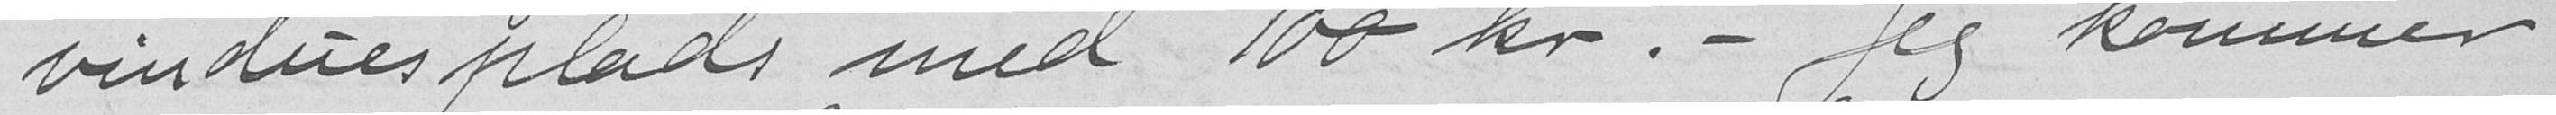vinduesplads med 100 kr. - Jeg kommer
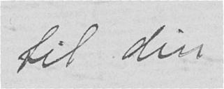til din
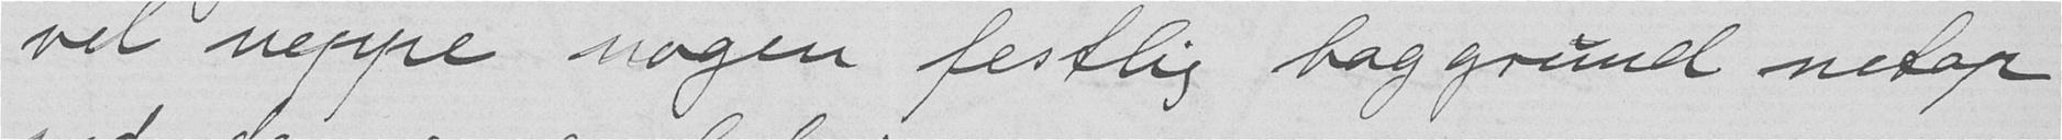vel neppe nogen festlig baggrund netop
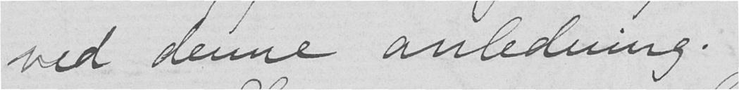ved denne anledning.
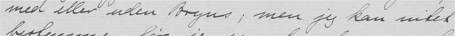med eller uden Bryns; men jeg kan intet
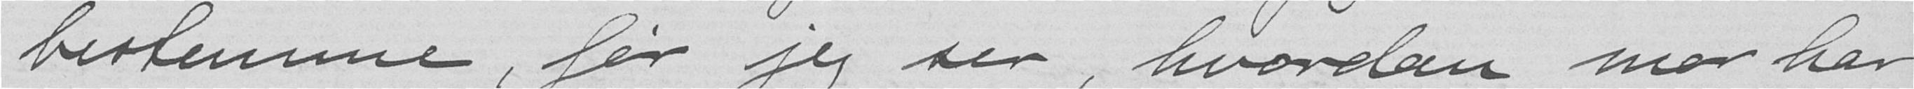bestemme, før jeg ser, hvordan mor har
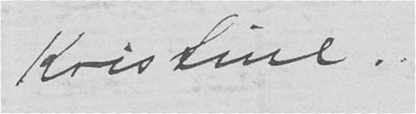Kristine.
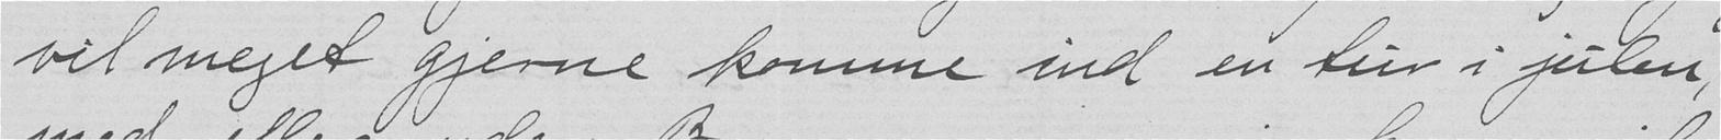vil meget gjerne komme ind en tur i julen,
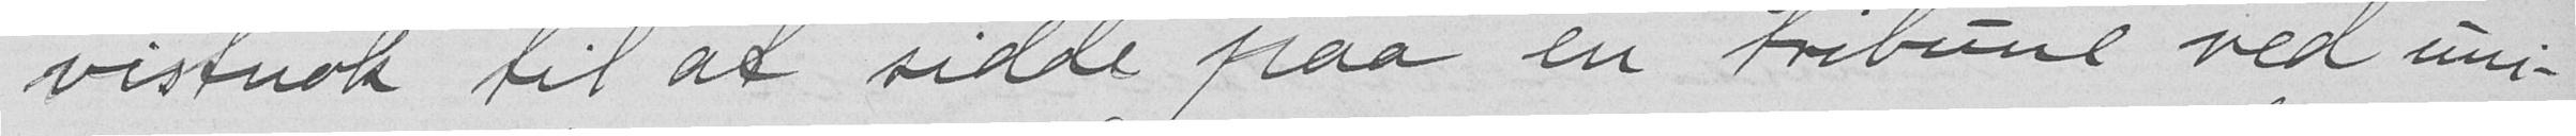vistnok til at sidde paa en tribune ved uni-
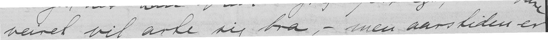veiret vil arte sig bra, - men aarstiden er
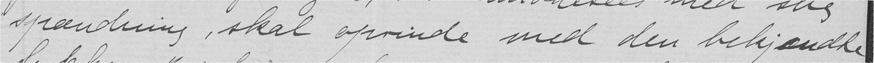spændning, skal oprinde med den bekjændte
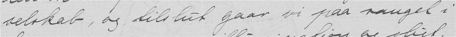selskab, og tilslut gaar vi paa rangel i
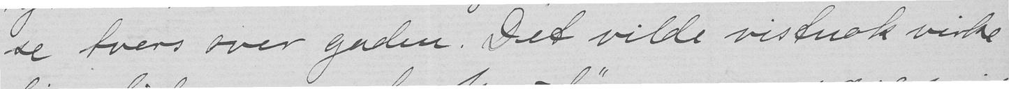se tvers over gaden. Det vilde vistnok virke
